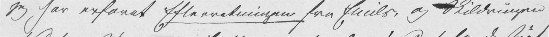jeg har erfaret Efterretningen fra Emils, og Skildringen
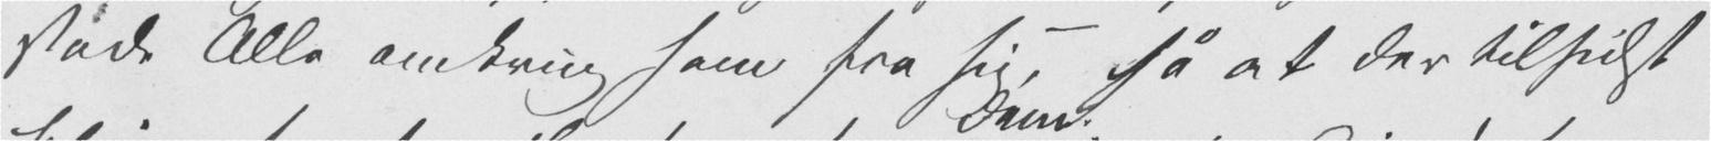støde Alle omkring ham fra sig, så at der tilsidst
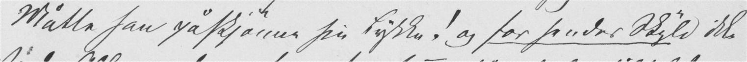Måtte han påskjønne sin Lykke! og for hendes Skyld ikke
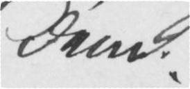dem
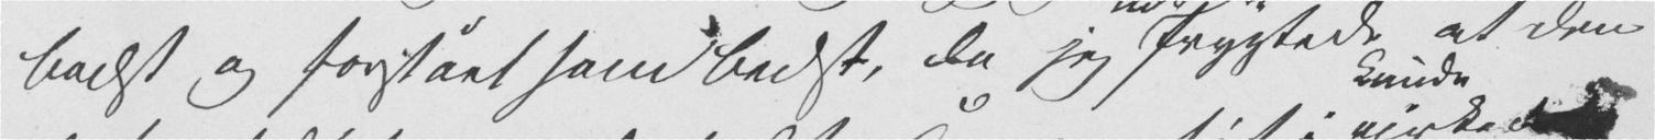bedst og forstået ham bedst, da jeg frygtede at den
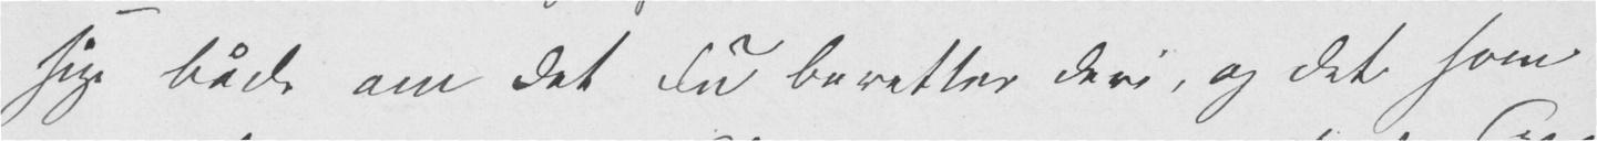sige både om det Du beretter deri, og det som
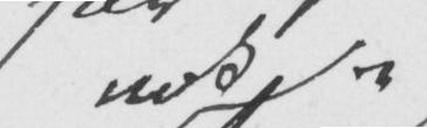nok
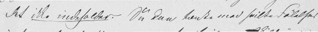det ikke indeholder. Du kan tænke med hvilke Følelser
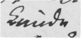kunde
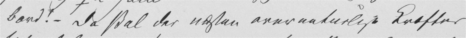Bord! – Da skal der næsten overnaturlige Kræfter
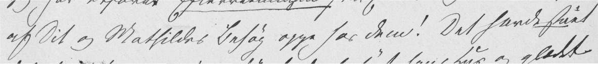af Dit og Mathildes Besøg oppe hos dem! Det havde stået
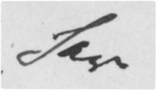har
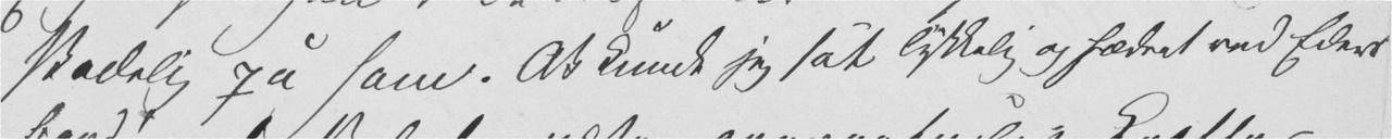skadelig på ham. Ak kunde jeg sat lykkelig og hædret ved Eders
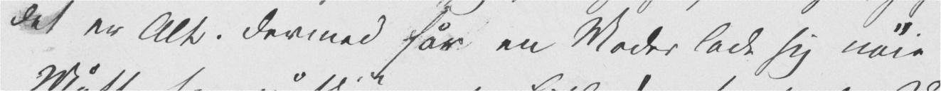det er Alt. Dermed har en Moder lade sig nøie
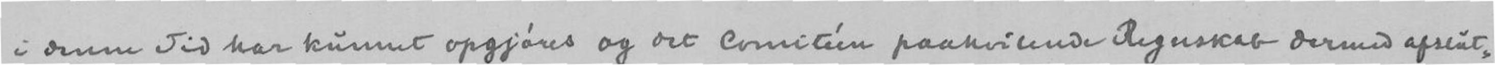i denne Tid har kunnet opgjøres og det Comitéen paahvilende Regnskab dermed afslut-
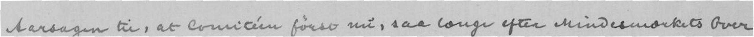Aarsagen til, at Comitéen først nu, saa længe efter Mindesmærkets Over-
